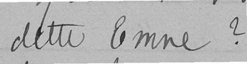dette Emne?
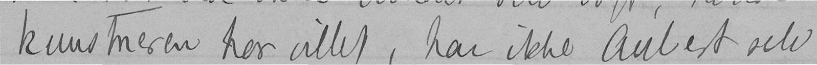kunstneren har villet, har ikke Aubert selv
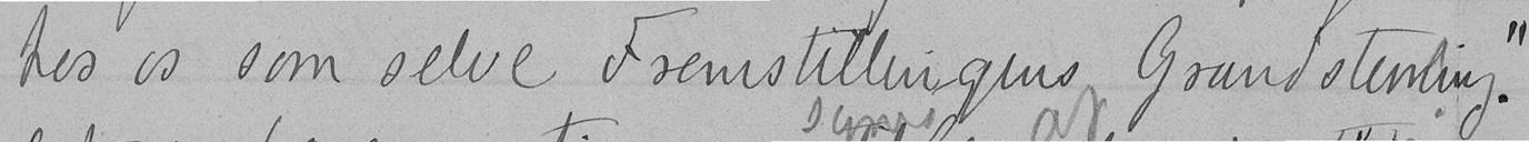hos os som selve Fremstillingens Grundstemning."
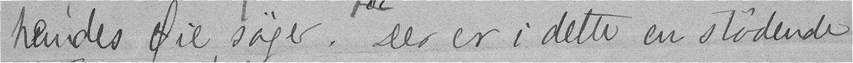hendes Øie søger. Der er i dette en stødende
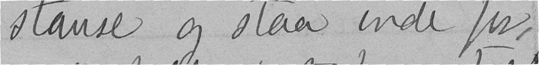stanse og staa inde for,
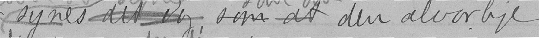synes det og, som at den alvorlige
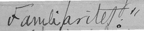Famliaritet."
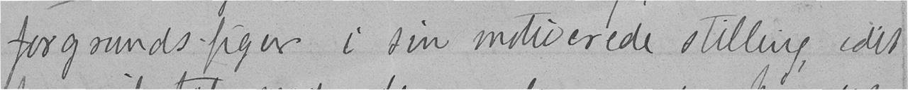forgrunds-figur i sin motiverede stilling, idet
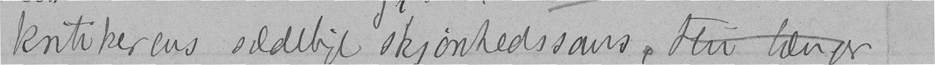kritikerens sædelige skjønhedssans, thi længer
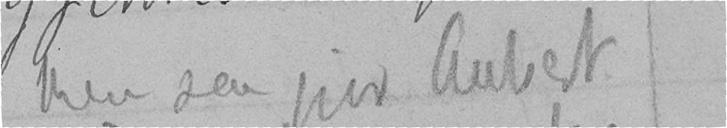Men saa sier Aubert
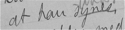at han synes,
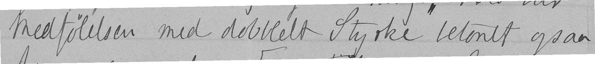medfølelsen med dobblelt Styrke betonet ogsaa
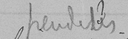pendelen
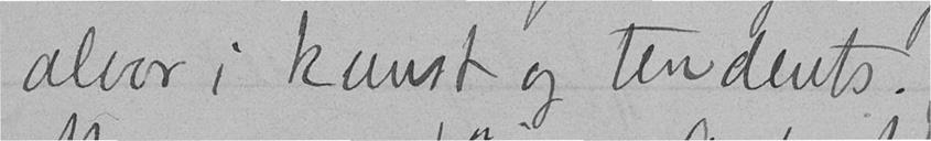alvor i kunst og tendents.
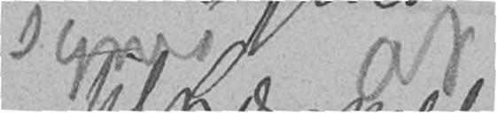synes at
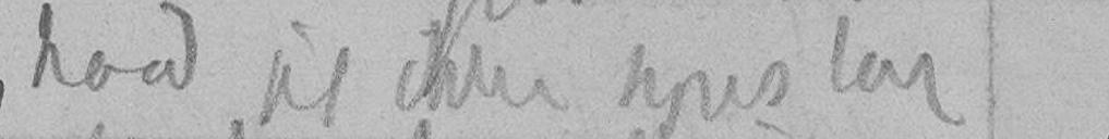hvad jeg ikke hos lar
